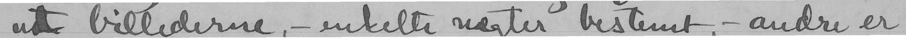ud billederne, - enkelte nægter bestemt, - andre er
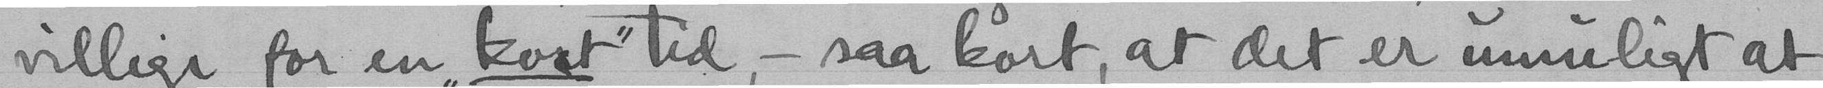villige for en "kort" tid, - saa kort, at det er umuligt at
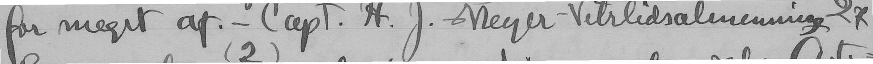for meget af. - Capt. H.J. Meyer Vetrlidsalmenning 27
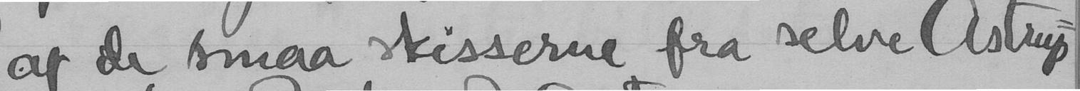af de smaa skisserne fra selve Astrup
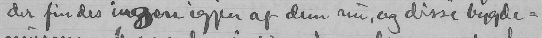der findes ingen igjen af dem nu, og disse bygde-
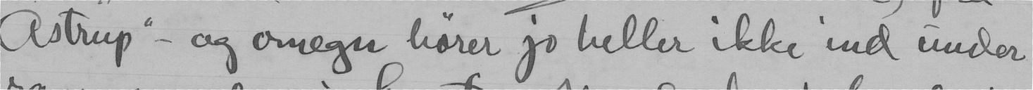"Astrup" - og omegn hører jo heller ikke ind under
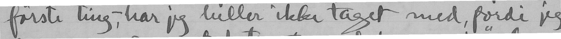første ting, har jeg heller ikke taget med, fordi jeg
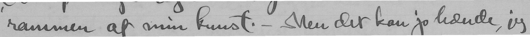rammen af min kunst. - Men det kan jo hænde, jeg
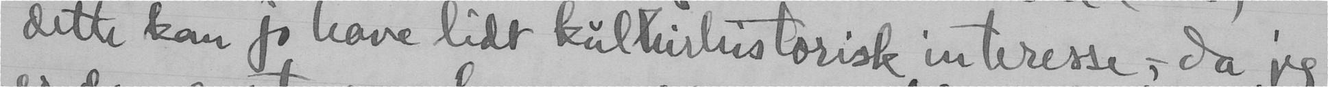dette kan jo have lidt kulturhistorisk interesse, - da jeg
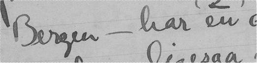Bergen - har en
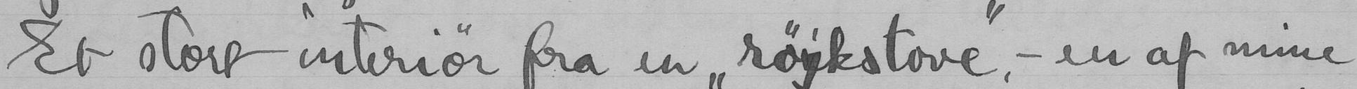Et stort interiør fra en "røykstove", - en af mine
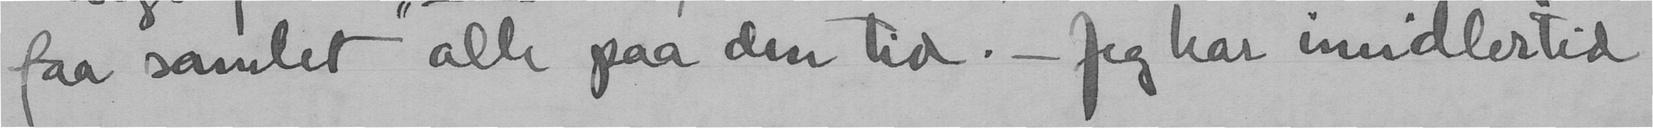faa samlet alle paa den tid. - Jeg har imidlertid
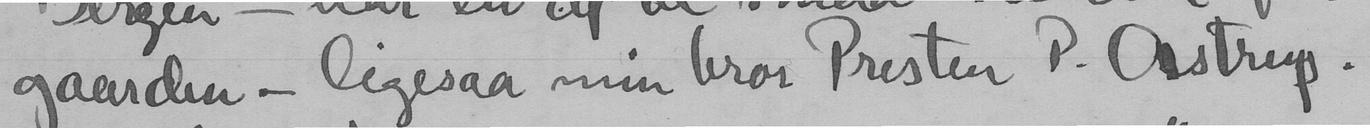gaarden - ligesaa min bror Presten P. Astrup.
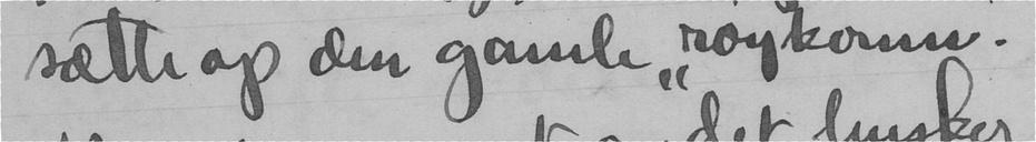sætte op den gamle "røykovn".
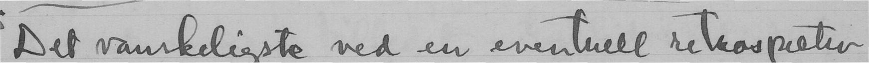Det vanskeligste ved en eventnell retaospectiv
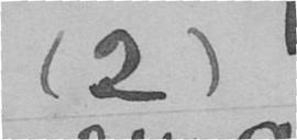(2)
